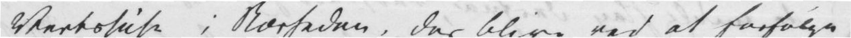Vertshuse i Nærheden, der blive ved at forfølge
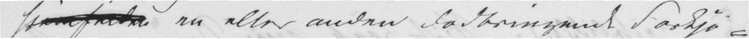hjem<gap /></del> en eller anden dødbringende Forkjø¬
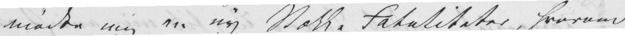mødte mig en ny Række Fataliteter, hvorom
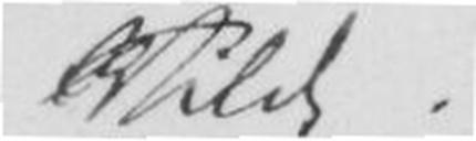Vilde.
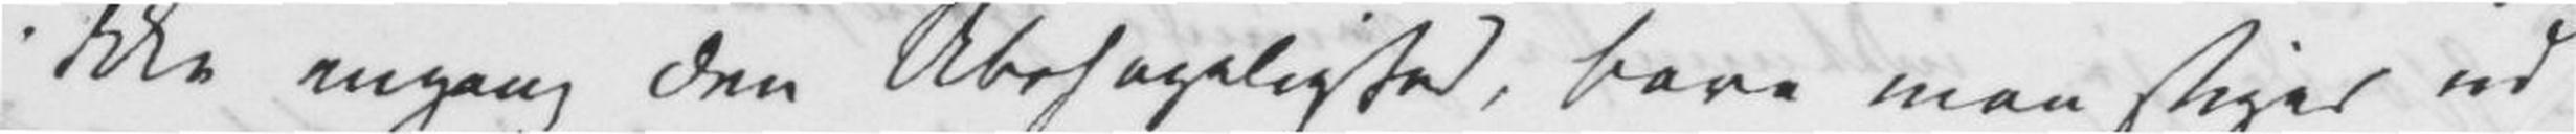ikke engang den Ubehagelighed, bare man stiger ud
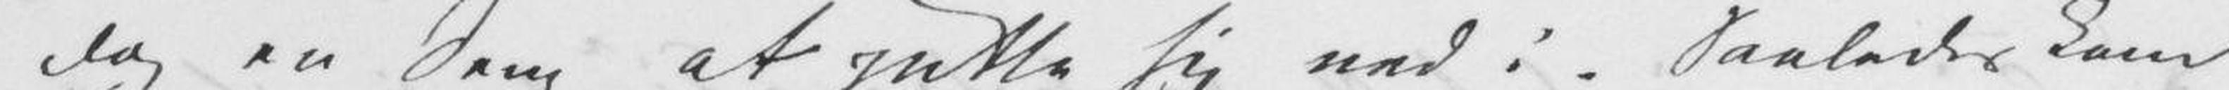dog en Seng at putte sig ned i. Saaledes kom
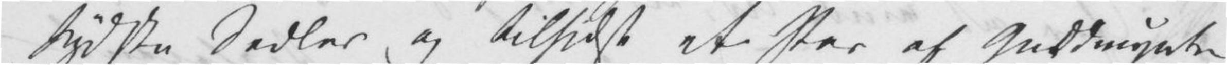tydske Sedler og tilsidst et Par af Guldmynter¬
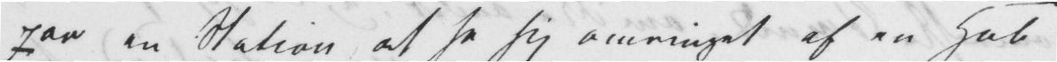paa en Station at se sig omringet af en Hob
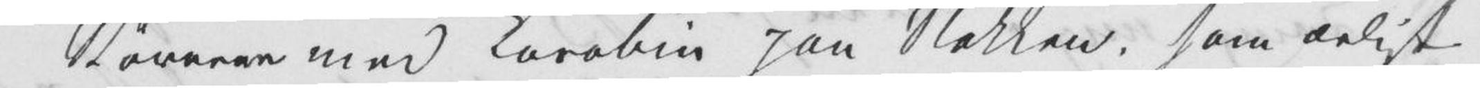Røveren med Karabin paa Nakken, som ærligt
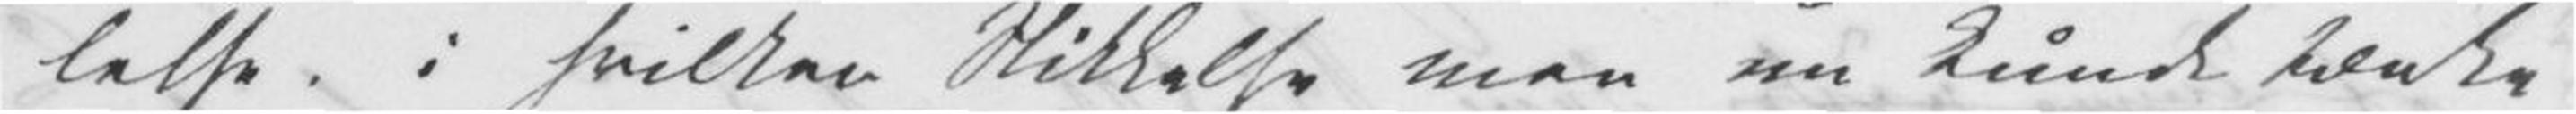lelse i hvilken Skikkelse man nu kunde tænke
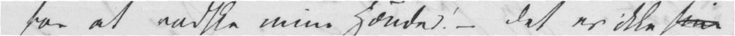for at vadske mine Hænder! – Det er ikke
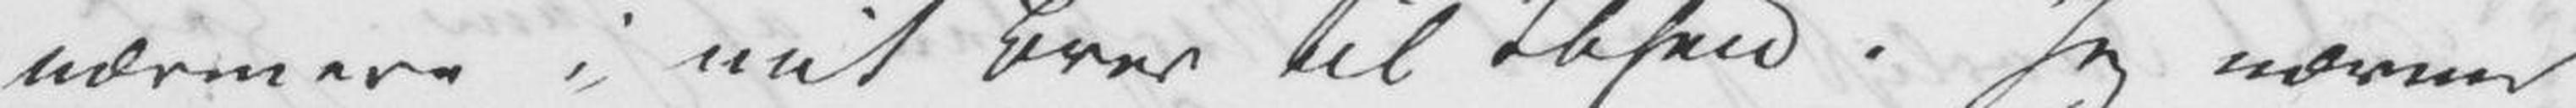nærmere i mit Brev til Ibsen. Jeg nævner
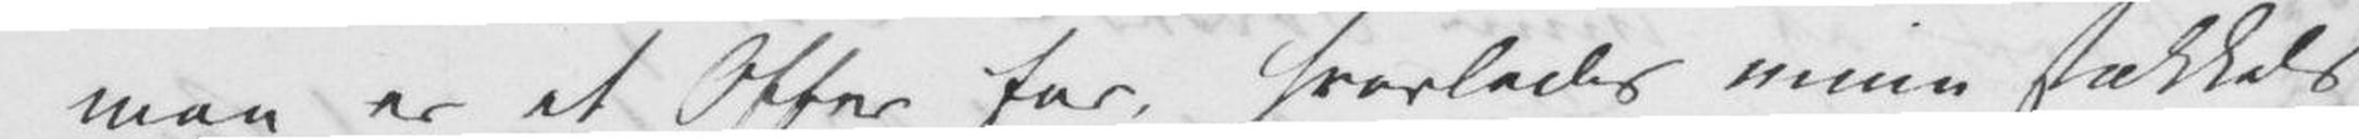man er et Offer for, hvorledes mine stakkels
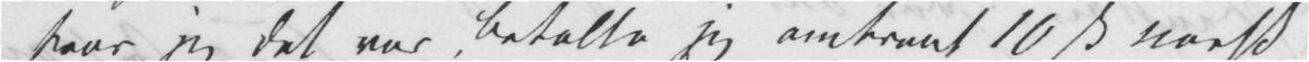tror jeg det var, betalte jeg omtrent 10 s[killing] norsk
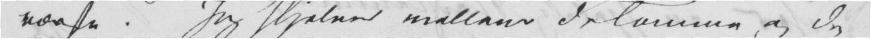værste. Jeg skjelner mellem de Tamme og de
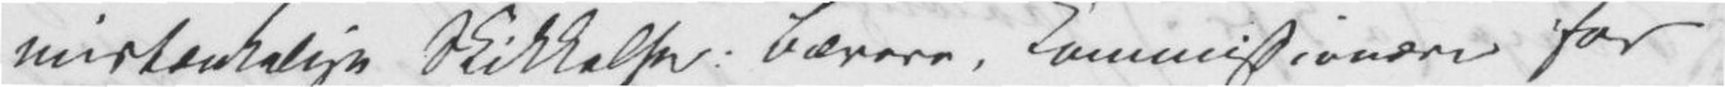mistænkelige Skikkelser: Bærere, Kommissionære for
